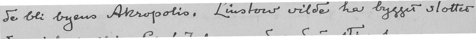de bli byens Akropolis. Lustow vilde ha bygget slottet
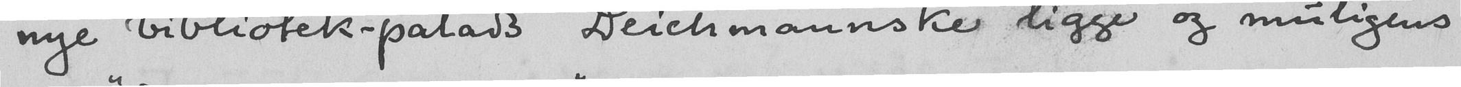nye bibliotek-palads "Deichmannske" ligge og muligens
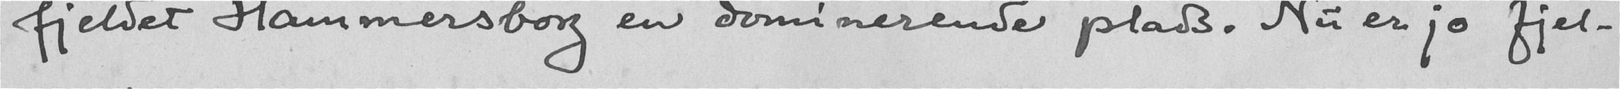fjeldet Hammersborg en dominerende plads. Nu er jo fjel-
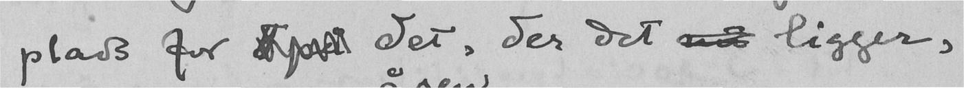plads for dpt det, der det nu ligger,
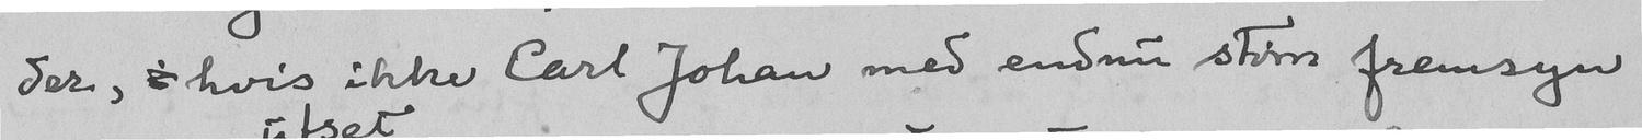der, i hvis ikke Carl johan med endnu større fremsyn
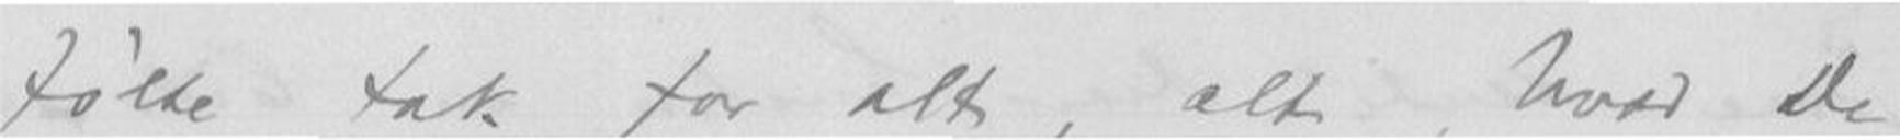følte tak for alt, alt, hvad De
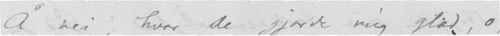Å nei, hvor De gjorde mig glad, o,
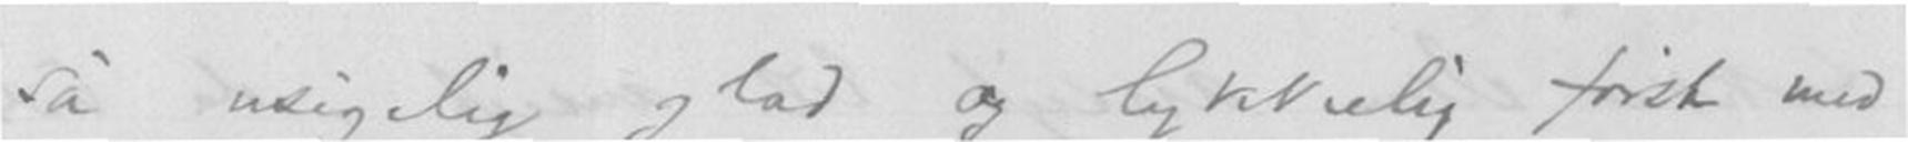så usigelig glad og lykkelig først med
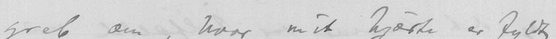greb om, hvor mit hjærte er fyldt
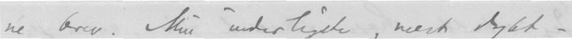ne brev. Min underligste, mest dybt-
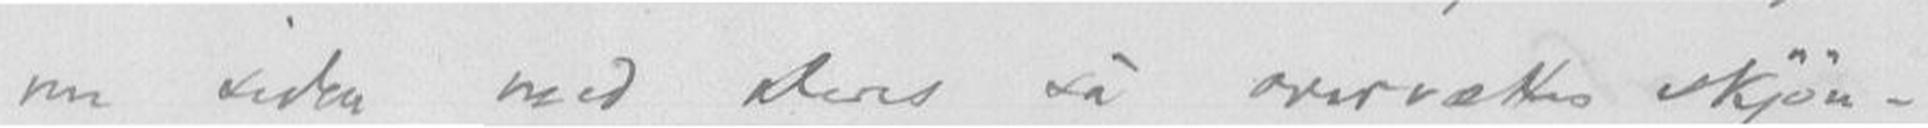nu siden med Deres så overvættes skjøn-
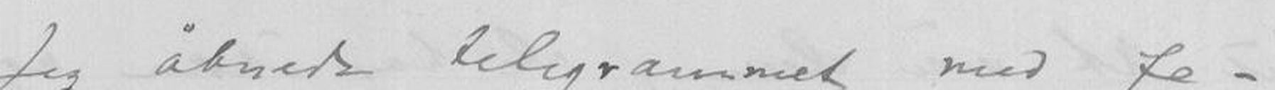Jeg åbnede telegrammet med fe-
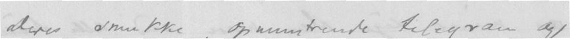Deres smukke, opmuntrende telegram og
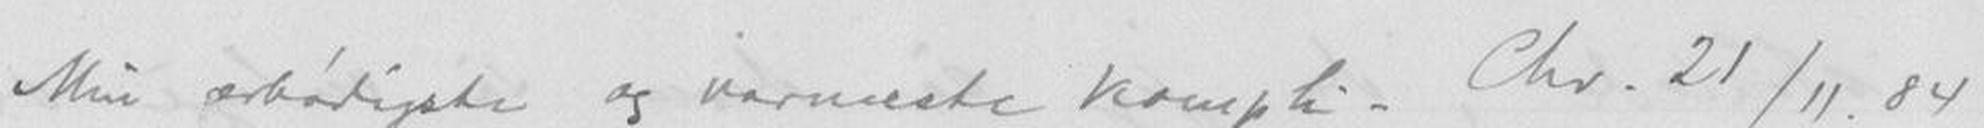Min ærbødiste og varmeste kompli- Chr. 21/11.84
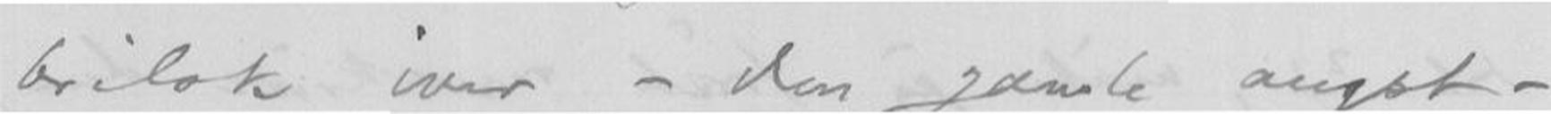brislk iver - den gamle angst -
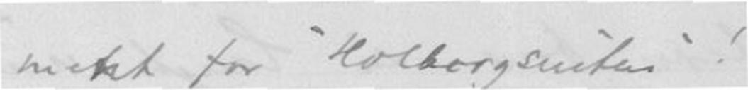mentet for "Holbergsuiten"!
